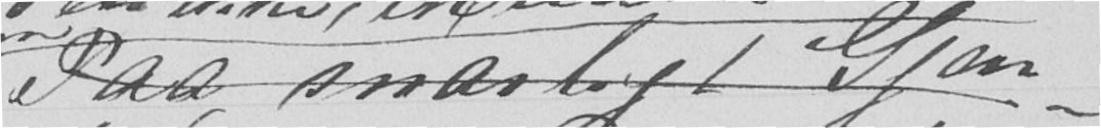Paa snarligt Gjen-
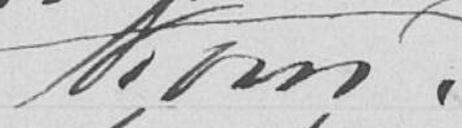kom,
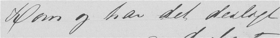Rom og har det deiligt
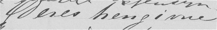Deres hengivne
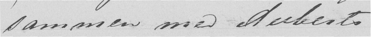sammen med Auberts
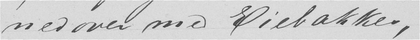nedover med Eiebakken,
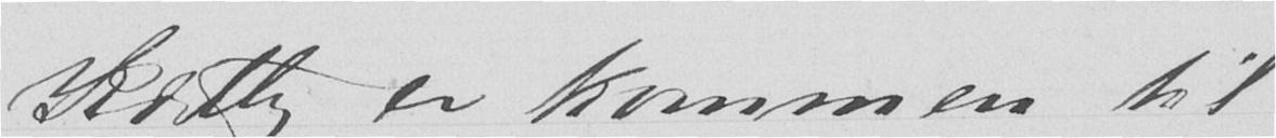Kitty er kommen til
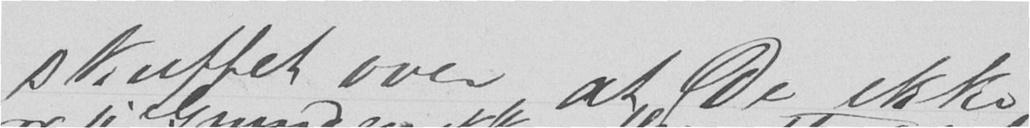skuffet over at De ikke
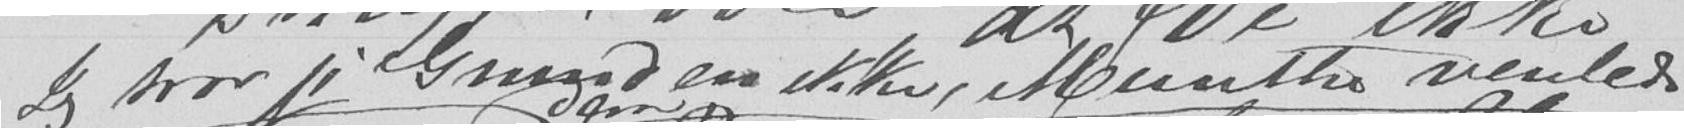Jeg tror i Grunden ikke, Munthe ventede
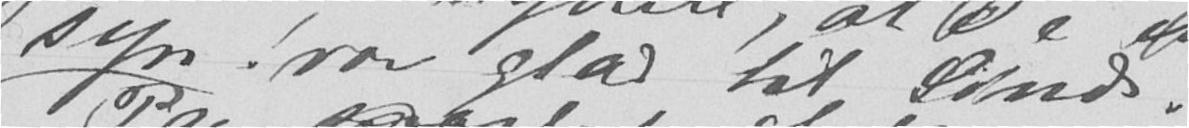syn! vær glad til Sinds.
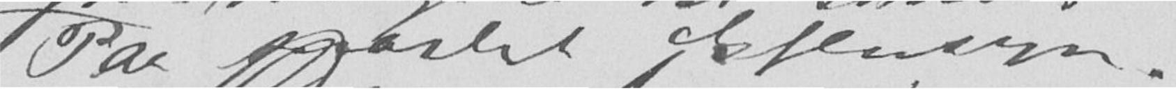Paa snarligt Gjensyn.
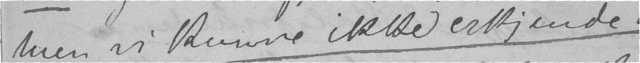Men vi kunne ikke erkjende at
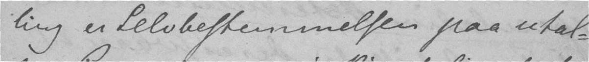ling er Selvbestemmelsen paa utal-
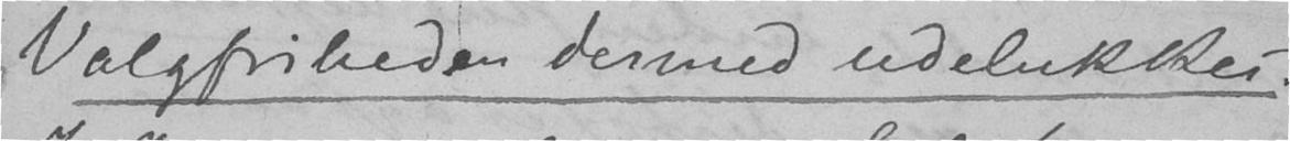Valgfriheden dermed udelukkes.
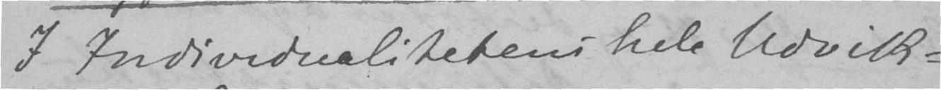I Individualitetens hele Udvik-
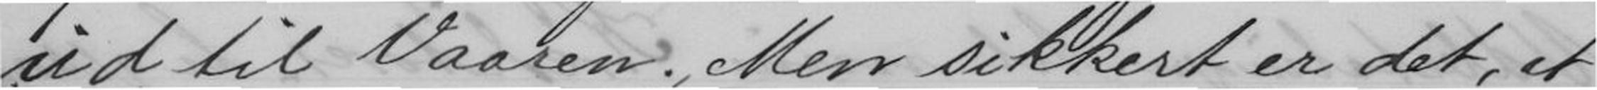ud til Vaaren. Men sikkert er det, et
